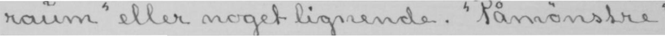raum» eller noget lignende. «Påmønstre»
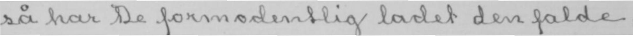så har De formodentlig ladet den falde,
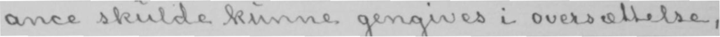ance skulde kunne gengives i oversættelse,
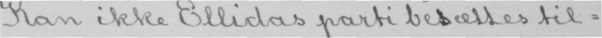Kan ikke Ellidas parti betsættes til-
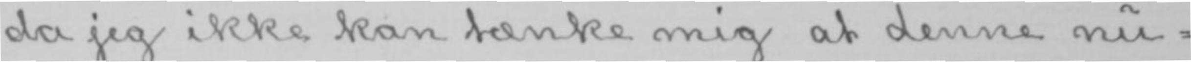da jeg ikke kan tænke mig at denne nu-
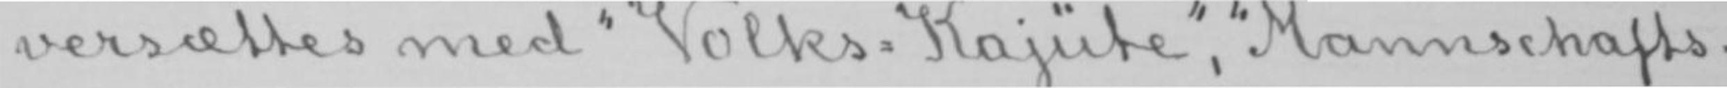versættes med «Volks-Kajüte», «Mannschafts-
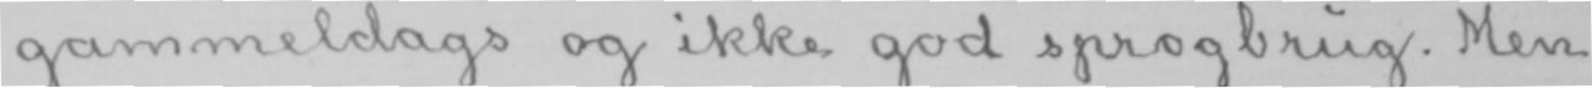gammeldags og ikke god sprogbrug. Men
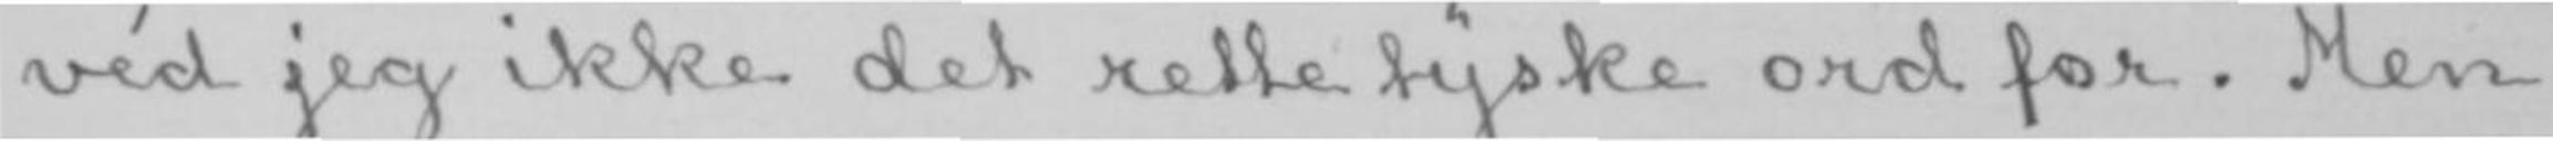véd jeg ikke det rette tyske ord for. Men
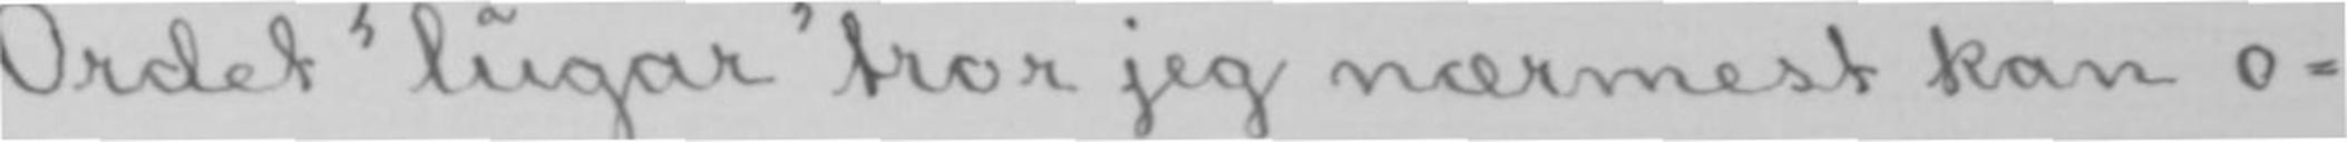Ordet «lugar» tror jeg nærmest kan o-
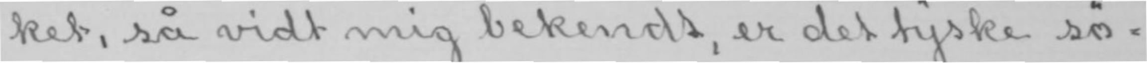ket, så vidt mig bekendt, er det tyske sø-
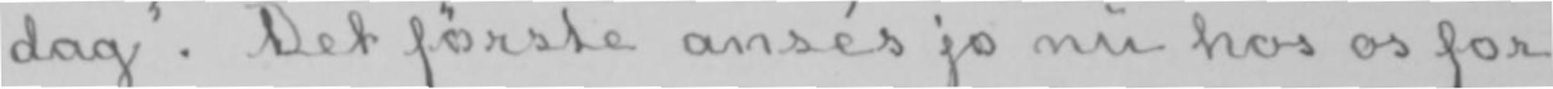dag». Det første ansés jo nu hos os for
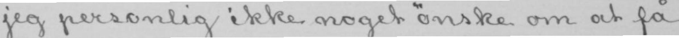jeg personlig ikke noget ønske om at få
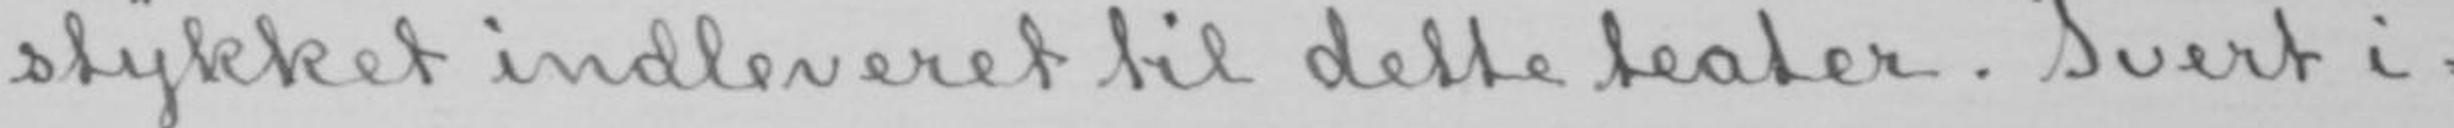stykket indleveret til dette teater. Tvert i-
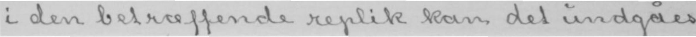i den betræffende replik kan det undgåes
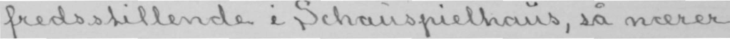fredsstillende i Schauspielhaus, så nærer
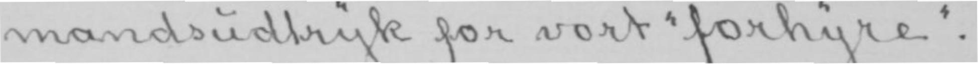mandsudtryk for vort «forhyre».-
